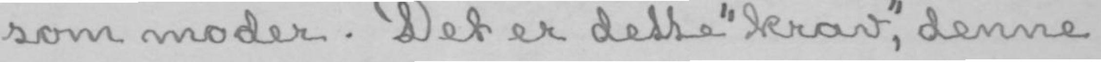som moder. Det er dette «krav», denne
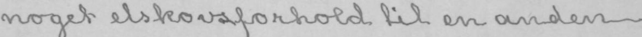noget elskovsforhold til en anden
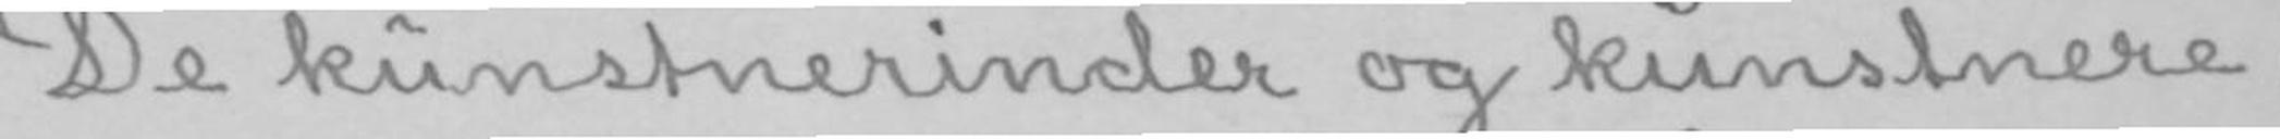De kunstnerinder og kunstnere,
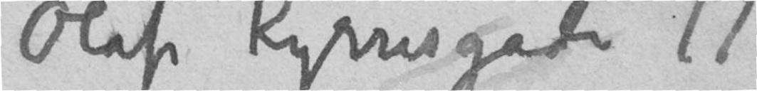Olaf Kyrresgade 11
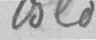Oslo
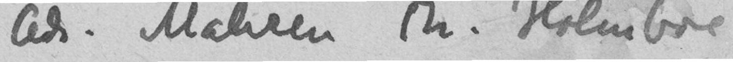Adr. Maleren Th. Holmboe
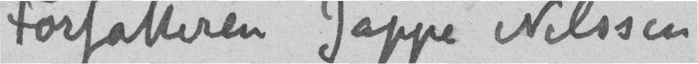Forfatteren Jappe Nilssen
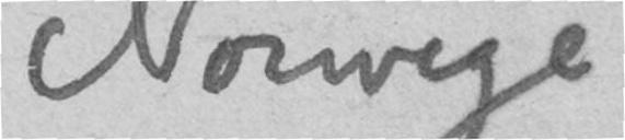Norwege
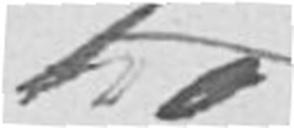tro
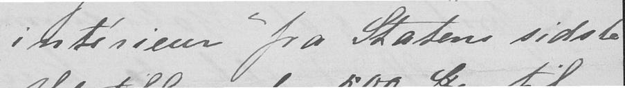interieur" fra Statens sidste
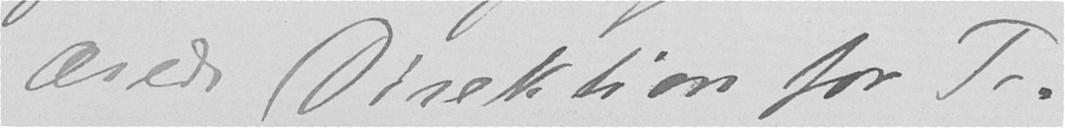ærede Direktion for Tr.
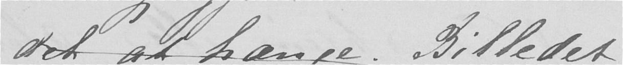det at hænge. Billedet
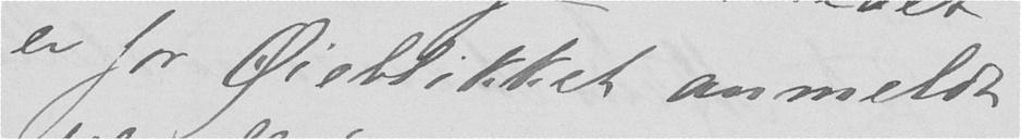er for Øieblikket anmeldt
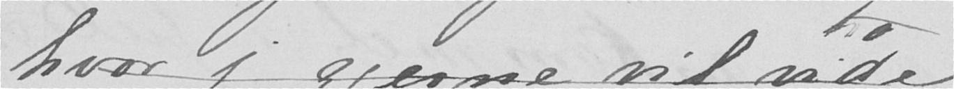hvor jeg gjerne vil vide
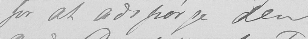for at udspørge den
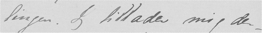lingen. Jeg tillader mig der-
